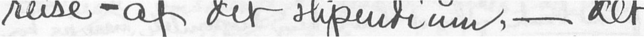reise - af dit stipendium, - det
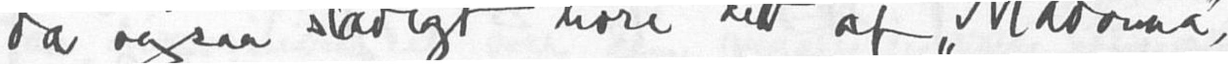da ogsaa stadigt høre det af "Madonna",
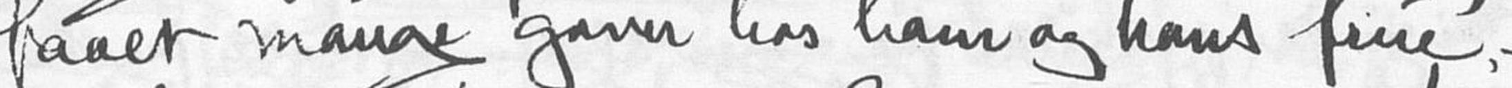faat mange gaver hos ham og hans frue, -
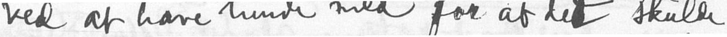ved at have hende med - for at det skulde
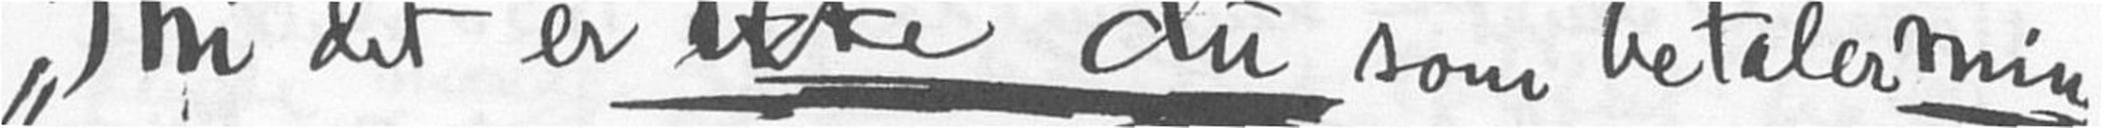"Thi det er ikke du som betaler min
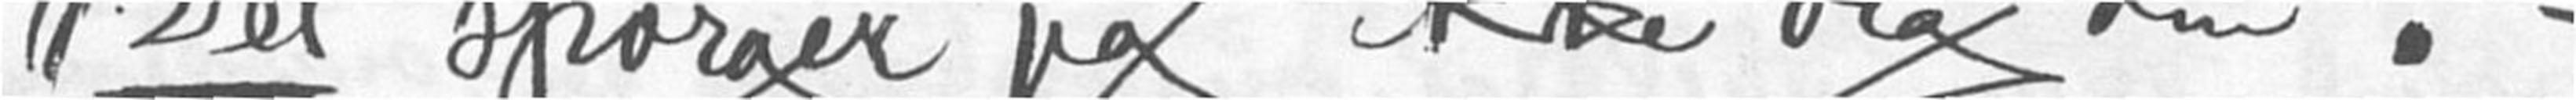Det spørger jeg ikke dig om!" -
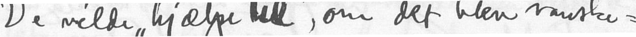De vilde "hjælpe til", om det blev vanske-
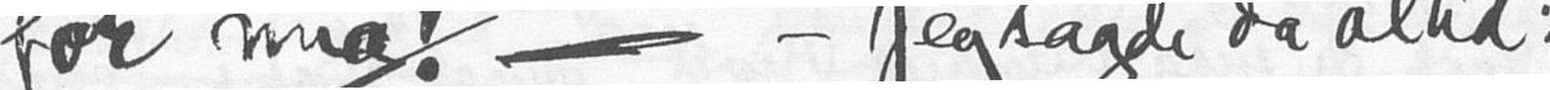for mig !" -Jeg sagde da altid:
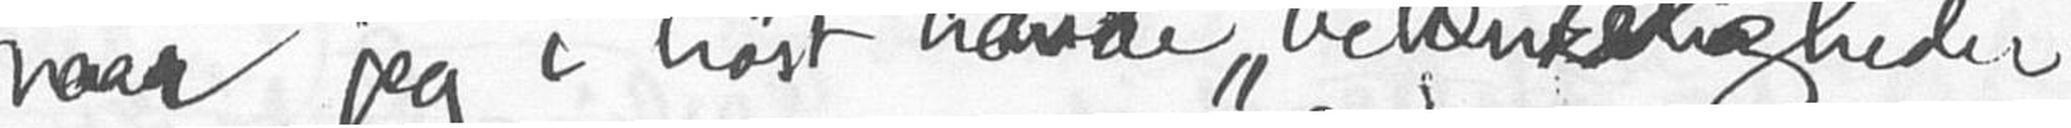naar jeg i høst havde "betænkeligheder"
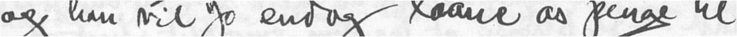og han vil jo endog laane os penge til
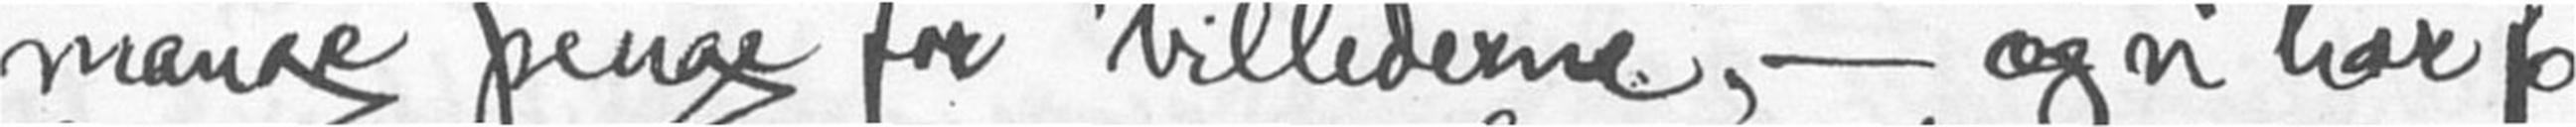mange penge for billederne, - og vi har jo
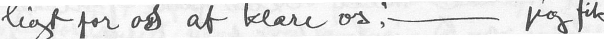ligt for os at klare os" - jeg fik
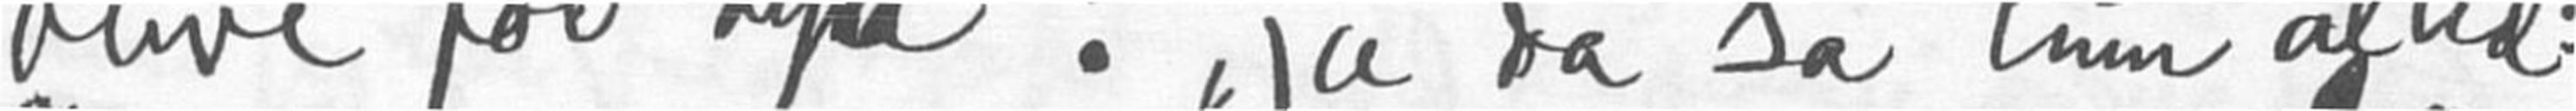blive for dyrt: "ja da sa hun altid:
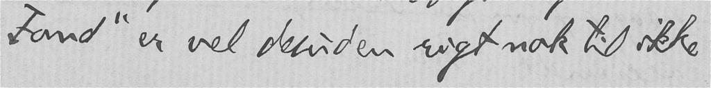Fond" er vel desuden rigt nok til ikke
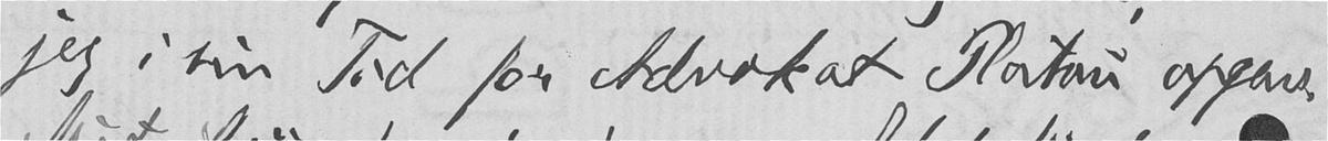jeg i sin Tid for Advokat Platou opgav.
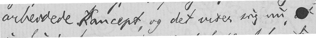arbeidede Koncept, og det viser sig nu, at
